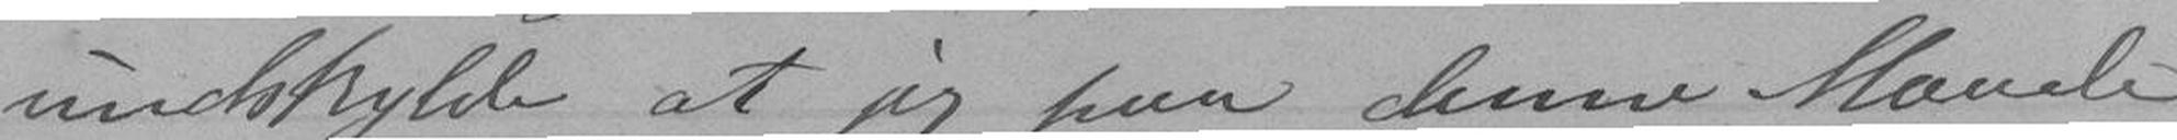undskylde at jeg paa denne Maade
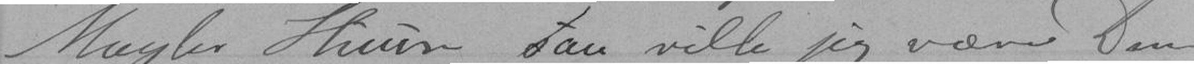Mægler Huuse saa ville jeg være Dem
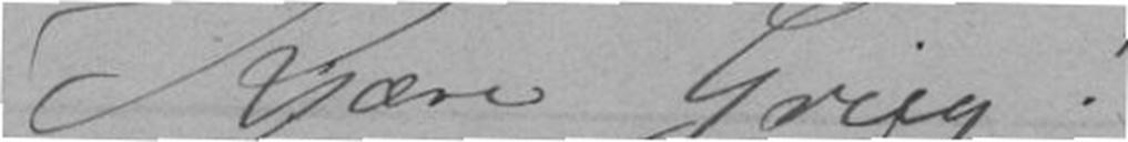Kjære Grieg!
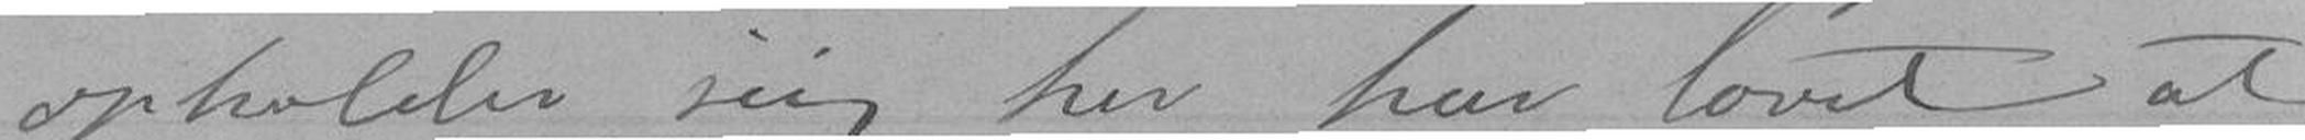opholder sig her har lovet at
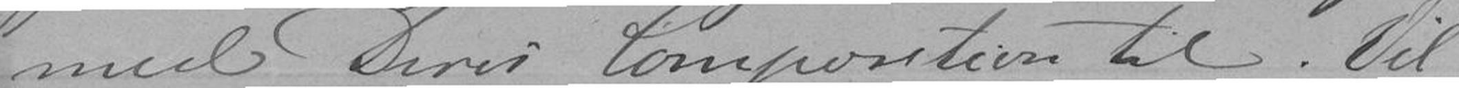med Deres Composition til. Vil
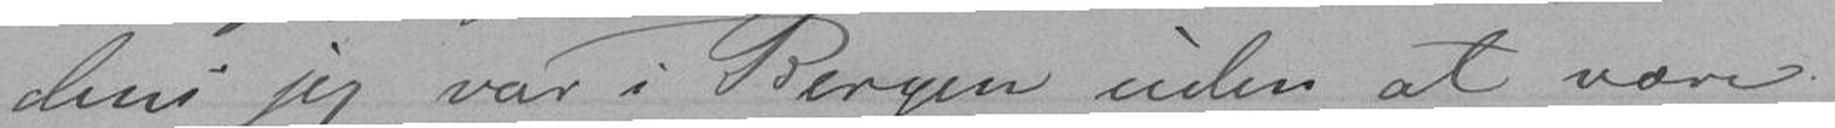dens jeg var i Bergen uden at være
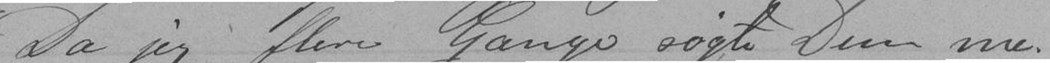Da jeg flere Gange søgte Dem me-
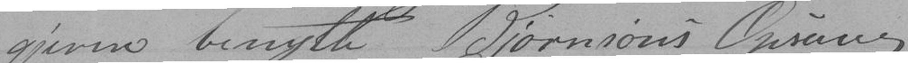gjerne benytte Bjørnsons Opsang,
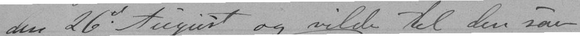den 26d August og vilde til den saa
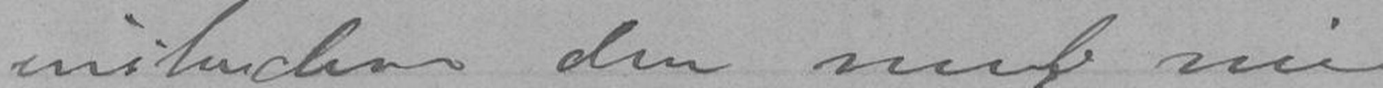instrudere den med mig
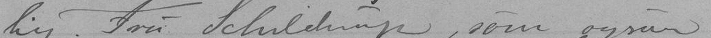lig. Fru Scheldrup, som ogsaa
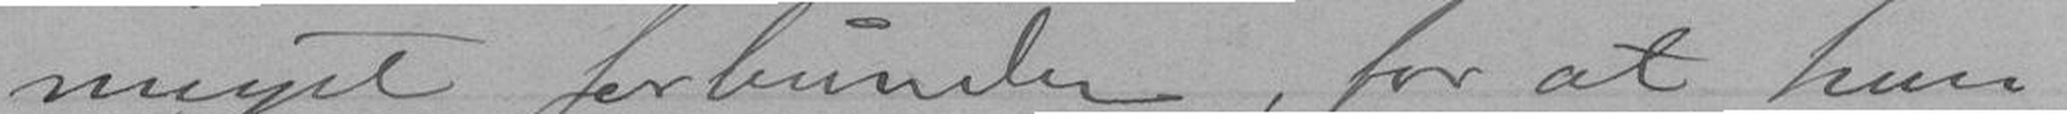meget forbunden, for at hun
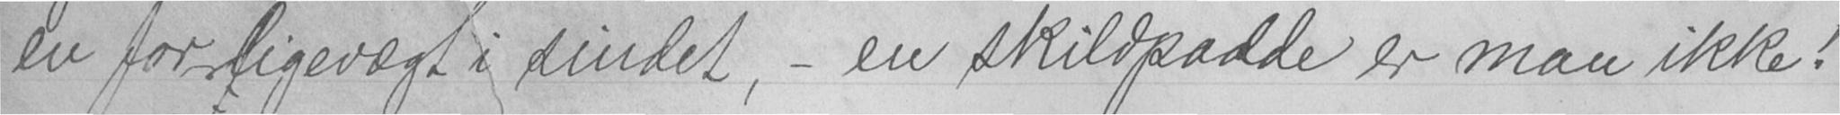en for ligevægt i sindet, - en skildpadde er man ikke!
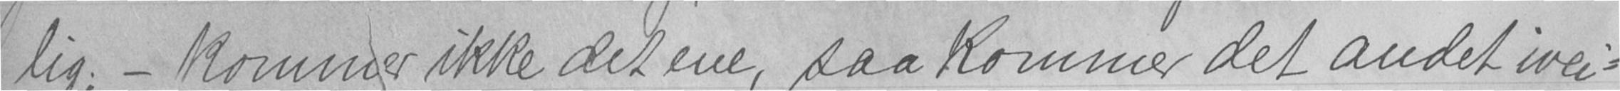lig; - kommer ikke det ene, saa kommer det andet ivei-
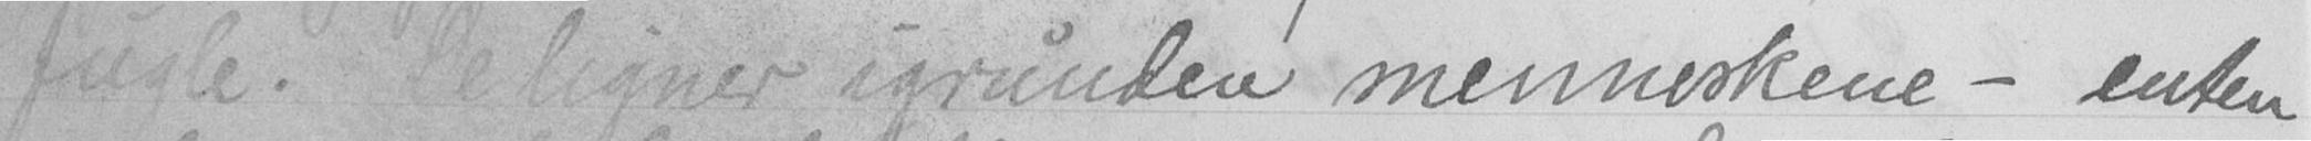fugle. De ligner igrunden menneskene - enten
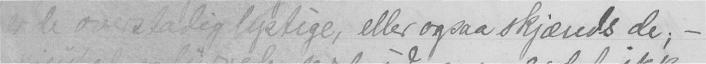er de overstadig lystige, eller ogsaa skjænds de; -
